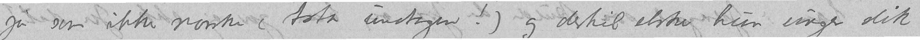ja som ikke norske (Asta undtagen!) og dertil elsker hun unger slik
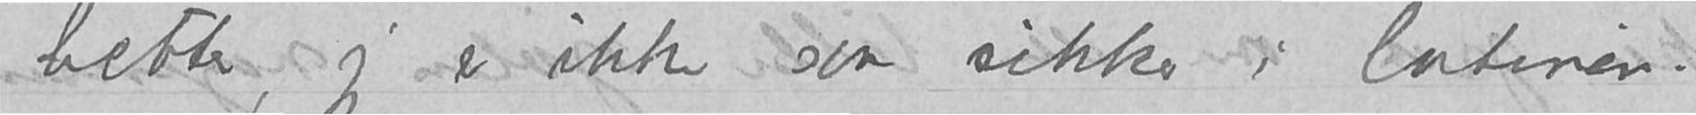hette, jeg er ikke saa sikker i latinen.
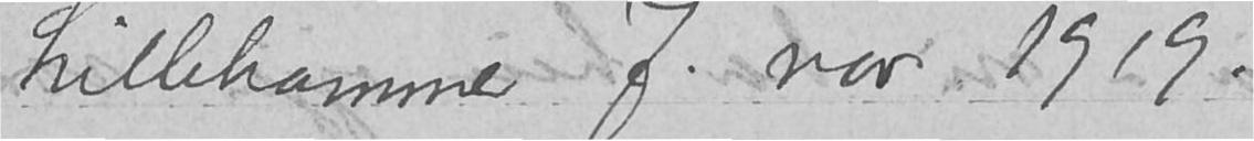Lillehammer 7. nov 1919.
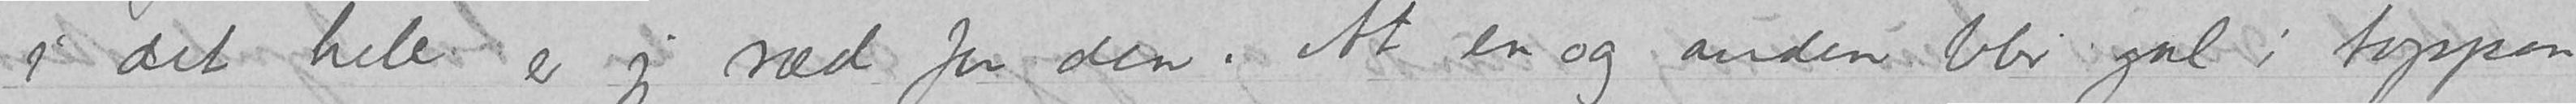i det hele er jeg ræd for den. At en og anden blir gal i toppen
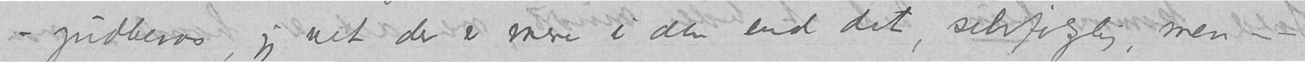– gudbevars, jeg vet der er mere i den end det, selvfølgelig, men –
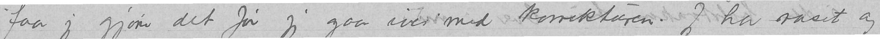faar jeg gjøre det før jeg gaar ivei med korrekturen. Jeg har raset og
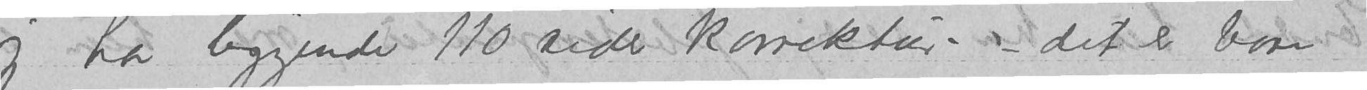jeg har liggende 110 sider korrektur: – det er bare
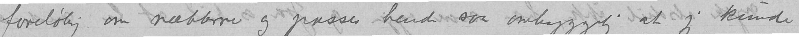foreløbig om nætterne og passer hende saa omhyggelig at jeg kunde
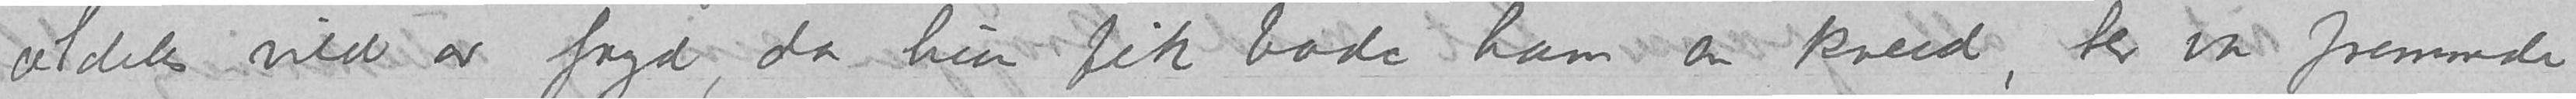aldeles vild av fryd, da hun fik bade ham en kveld, her var fremmede
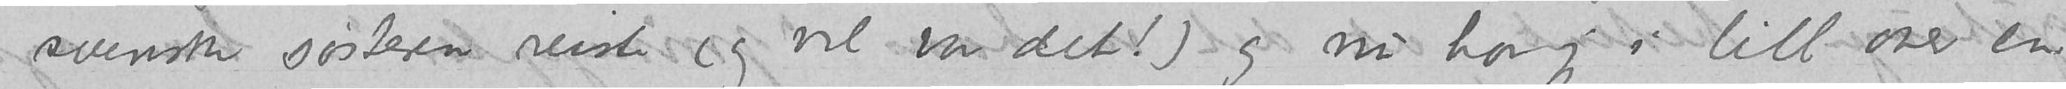svenske søsteren reiste (og vel var det!) og nu har jeg i litt over en
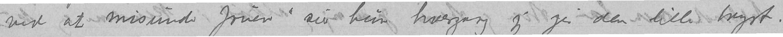ved at misunde fruen» sier hun hvergang jeg gir den lille bryst.
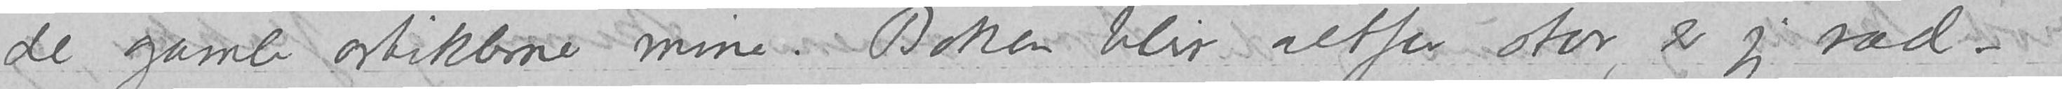de gamle artiklerne mine. Boken blir altfor stor, er jeg ræd –
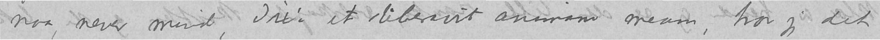naa, never mind, Dixi et liberavit animam meam, tror jeg det
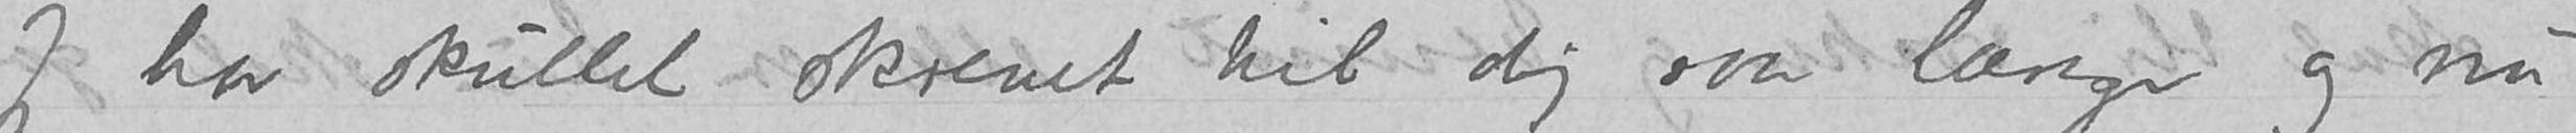Jeg har skullet skrevet til dig saa længe og nu
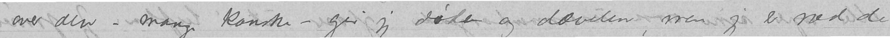over den – mange kanske – gir jeg døden og dævelen, men jeg er ræd de
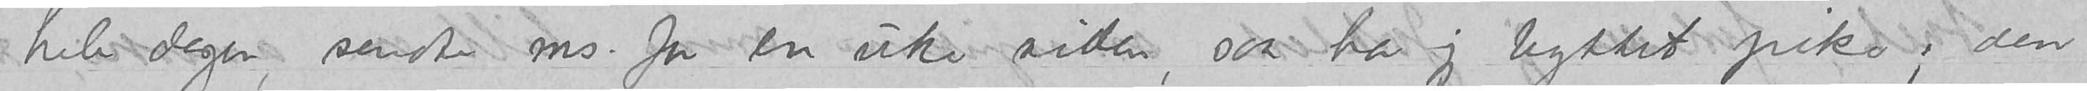hele dagen, sendte ms. for en uke siden, saa har jeg byttet pike; den
